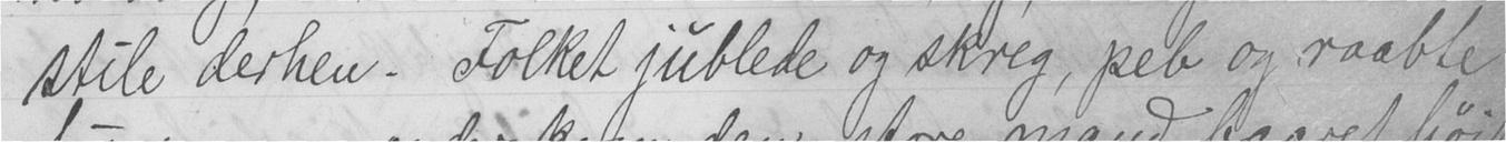stile derhen. Folket jublede og skreg, peb og raabte
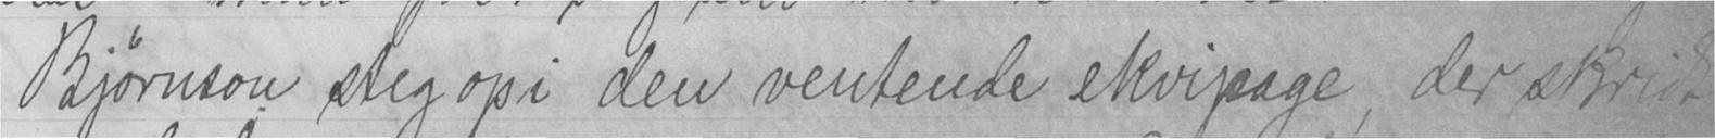Bjørnson steg opi den ventende ekvipage, der skridt
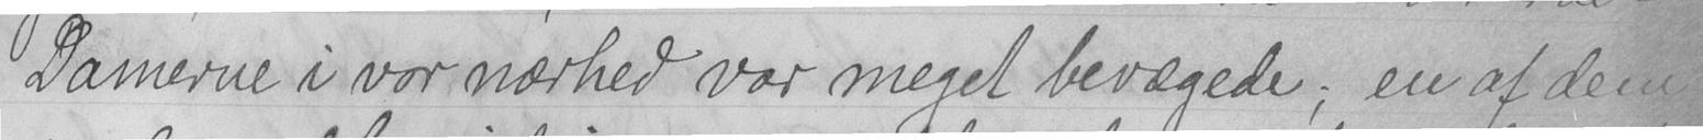Damerne i vor nærhed var meget bevægede; en af dem
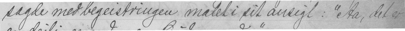sagde med begeistringen malet i sit ansigt: "Aa, det er
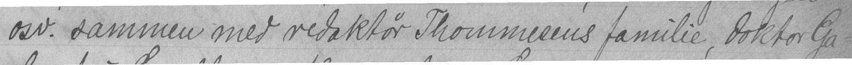osv. sammen med redaktør Thommesens familie, doktor Ga-
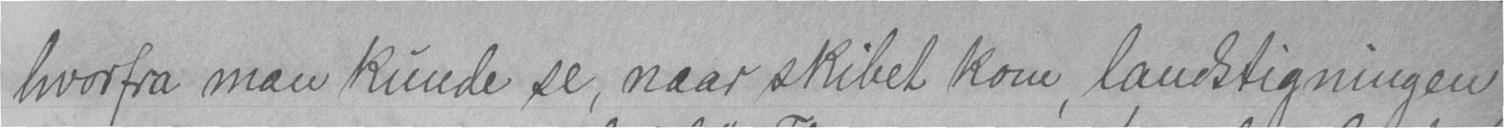hvorfra man kunde se, naar skibet kom, landstigningen
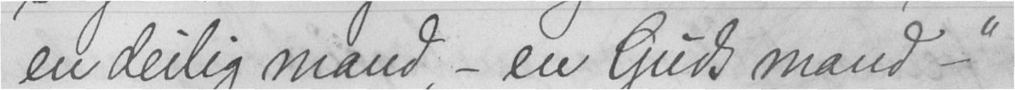en deilig mand, - en Guds mand - "
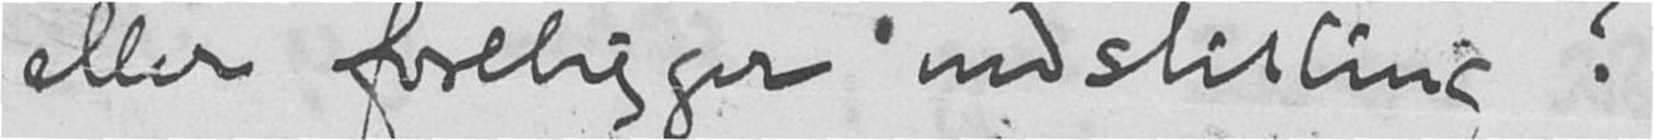eller foreligger indstilling?
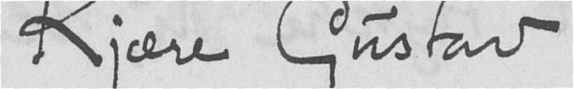Kjære Gustav
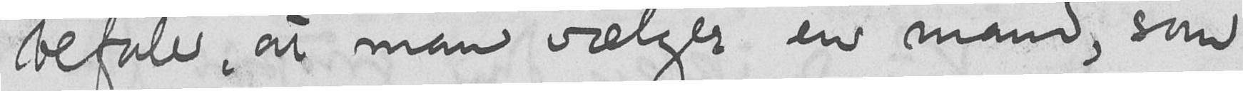befale, at man vælger en mand, som
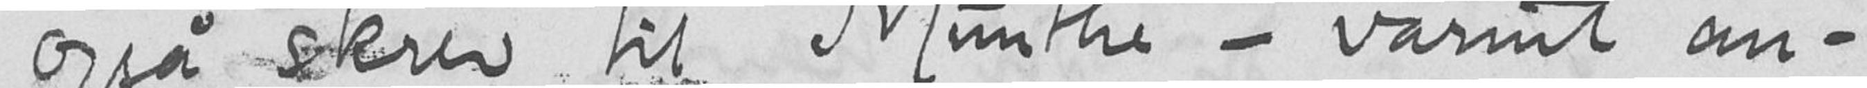også skrev til Munthe - varmt an-
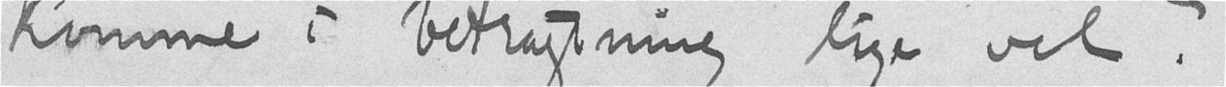komme i betragtning lige vel?
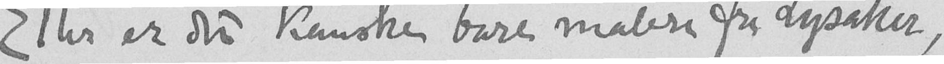Eller er det kanske bare malere fra Lysaker,
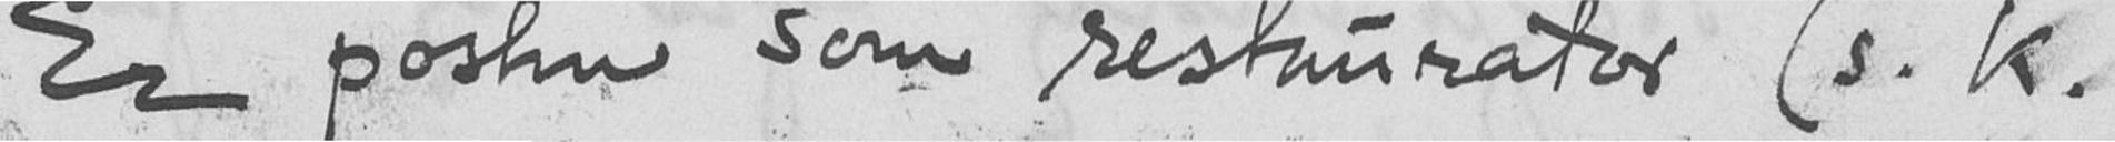Er posten som restaurator (s.k.
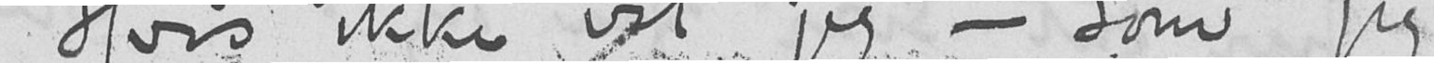Hvis ikke vil jeg - som jeg
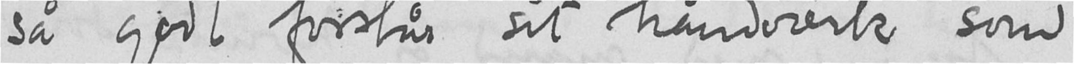så godt forstår sit håndværk som
